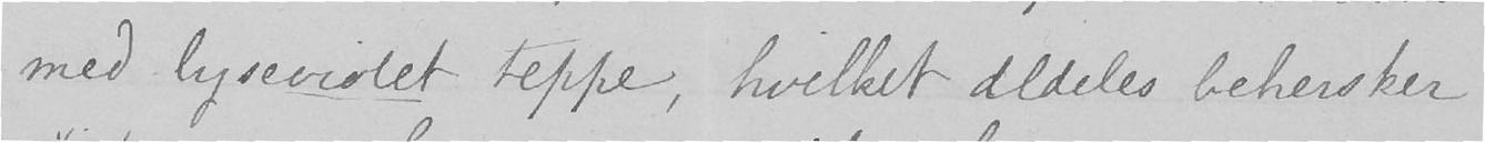med lyseviolet teppe, hvilket aldeles behersker
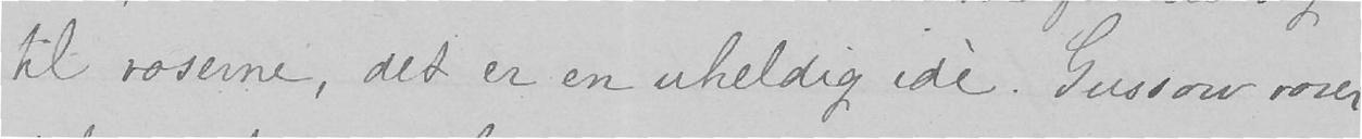til roserne, det er en uheldig idé. Gussow roser
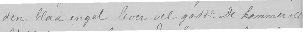den blaa engel lever vel godt? De kommer vel
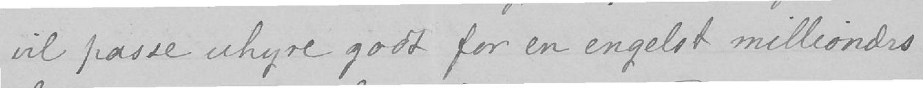vil passe uhyre godt for en engelsk millionærs
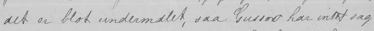det er blot undermalet, saa Gussow har intet sagt.
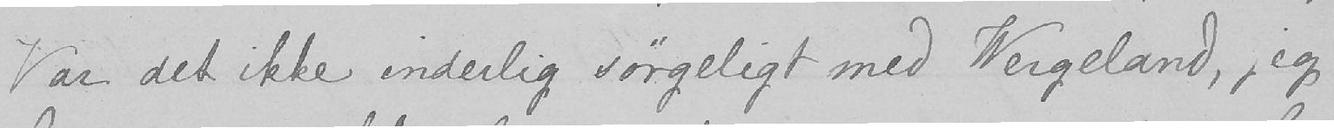Var det ikke inderlig sørgeligt med Wergeland, jeg
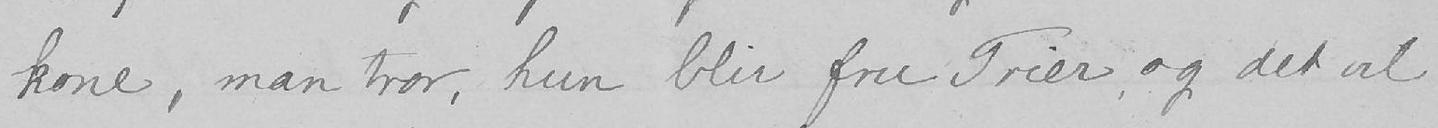kone, man tror, hun blir fru Trier, og det vil
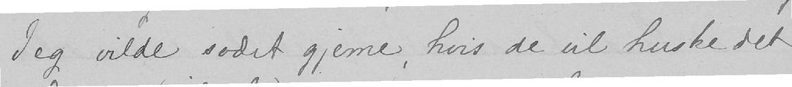Jeg vilde svært gjerne, hvis de vil huske det
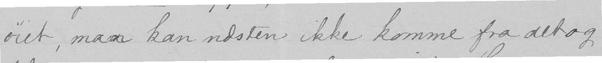øiet, man kan næsten ikke komme fra det og
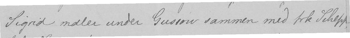Sigrid maler under Gussow sammen med frk Schepp
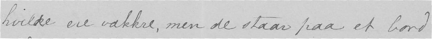hvilke ere vakkre, men de staar paa et bord
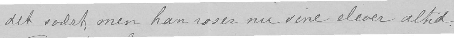det svært, men han roser nu sine elever altid.
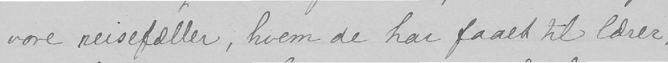vore reisefæller, hvem de har faaet til lærer,
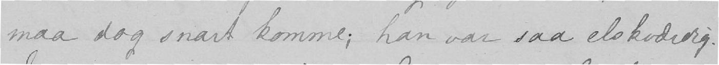maa dog snart komme; han var saa elskværdig.
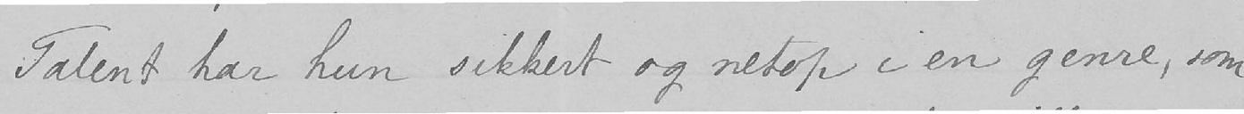Talent har hun sikkert og netop i en genre, som
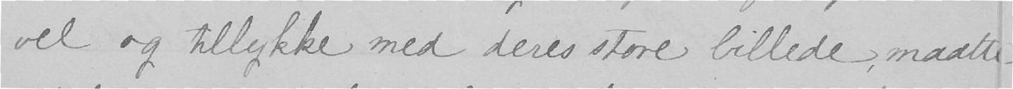vel og tillykke med deres store billede, maatte
